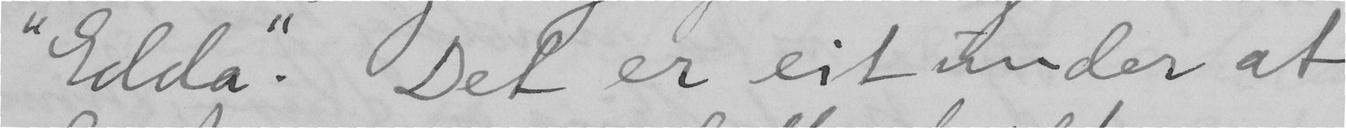"Edda". Det er eit under at
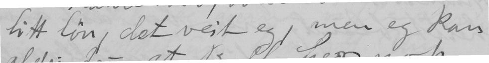litt löøn, det veit eg, men eg kan
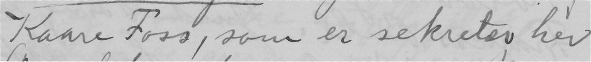Kaare Foss, som er sekretær hev
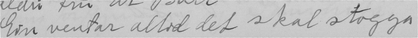Ein ventar altid det skal stogga
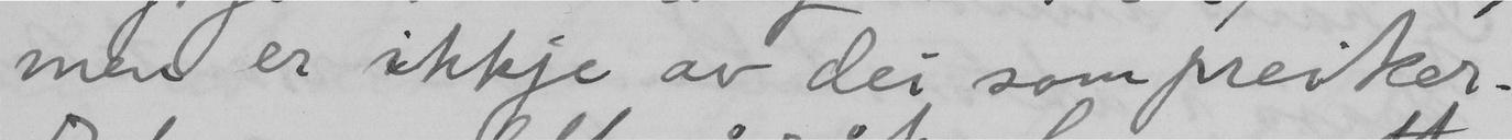men er ikkje av dei som preiker.
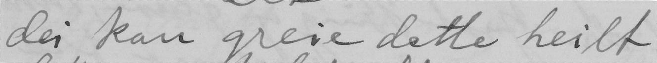dei kan greie dette heilt
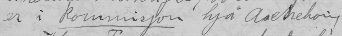er i kommisjon hjå Aschehoug
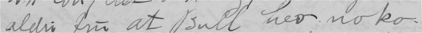aldri tru at Bull hev noko.
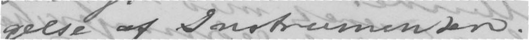gelse af Instrumenter.
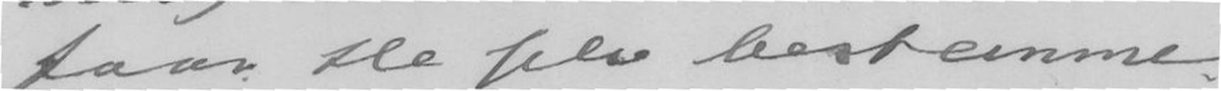faar De selv bestemme
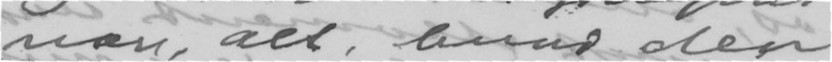være, alt hvad der
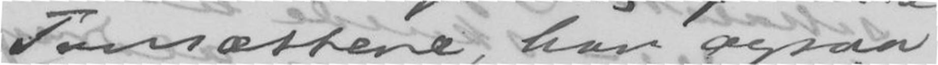Tonsættere, har ogsaa
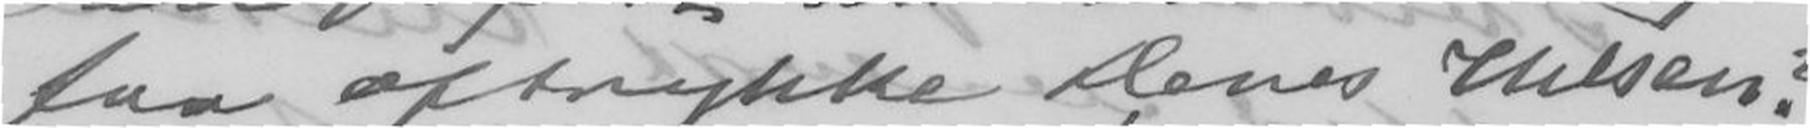faa aftrykke Deres Hilsen?
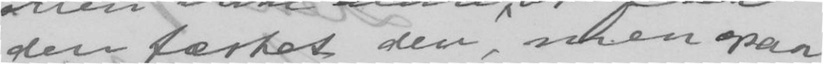den fæstet der, men opaa
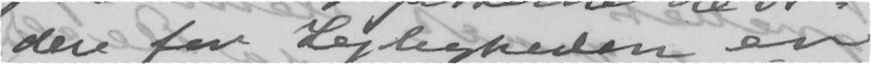der for Lejligheden er
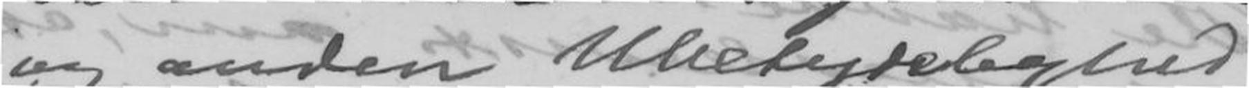og anden Ubetydelighed
